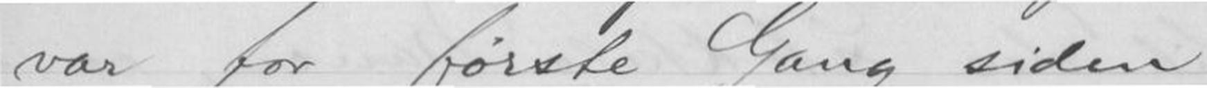var for første Gang siden
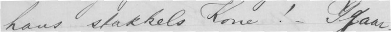hans stakkels Kone! - Igaar-
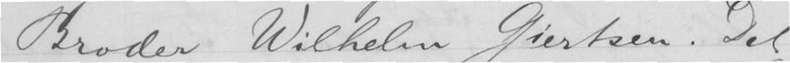Broder Wilhelm Giertsen. Det
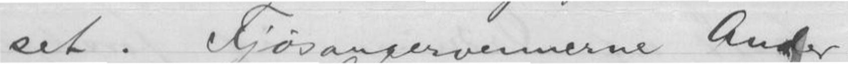set. Fjøsangervennerne Ander-
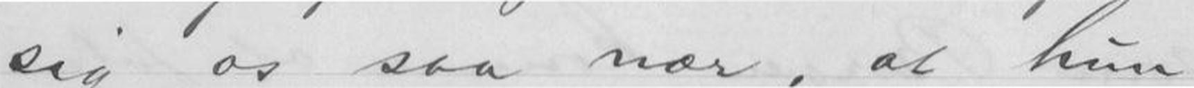sig os saa nær, at hun
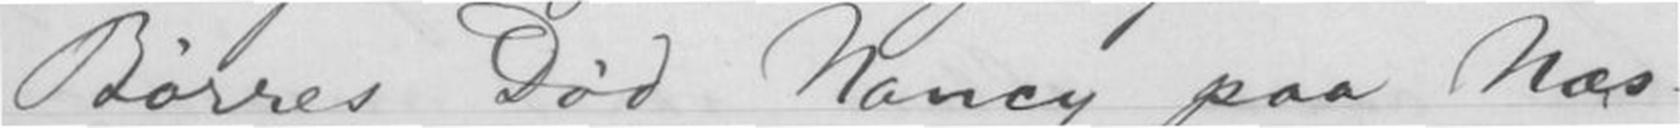Børres Død Nancy paa Næs-
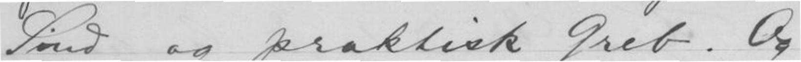Sind og praktisk Greb. Og
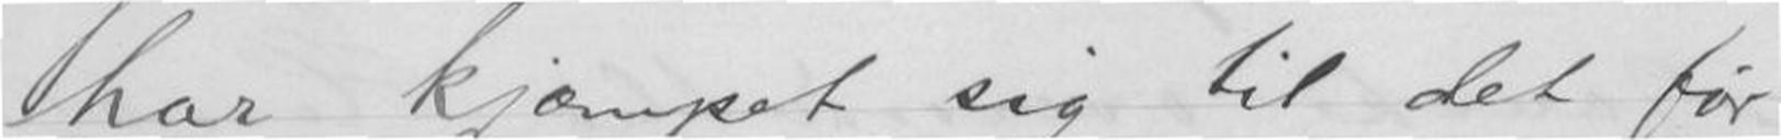har kjæmpet sig til det før-
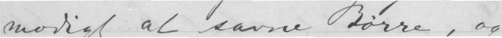modigt at savne Børre, og
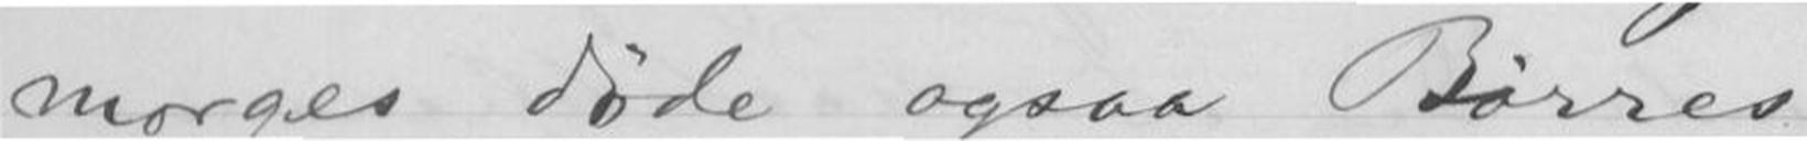morges døde ogsaa Børres
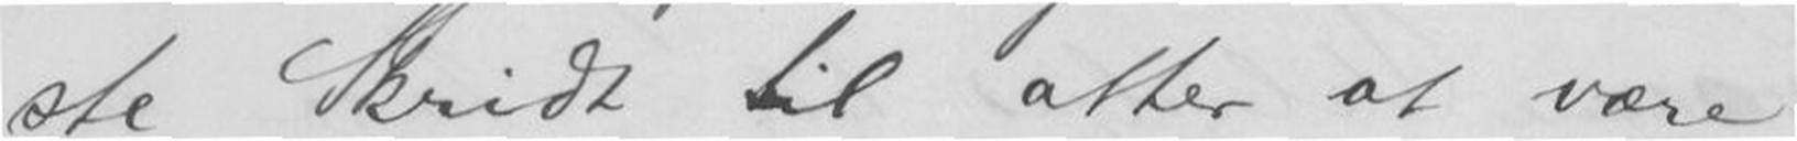ste Skridt til atter at være
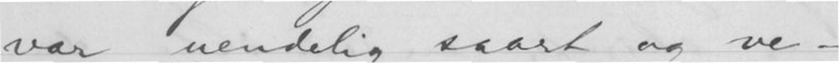var uendelig saart og ve-
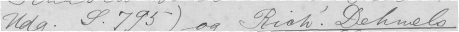Udg. S.795) og Rich. Dehmels
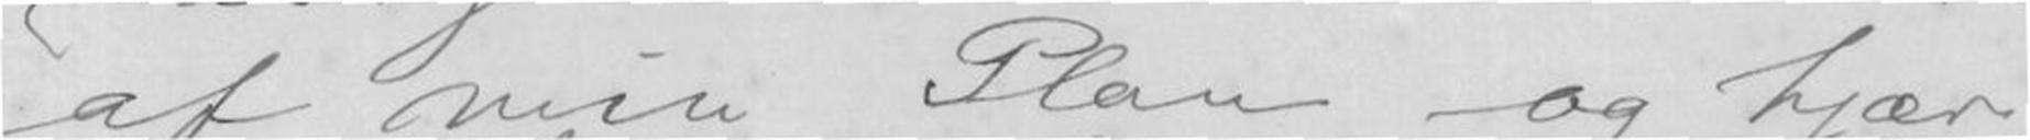af min Plan og hjær
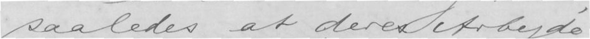saaledes at deres Arbeide
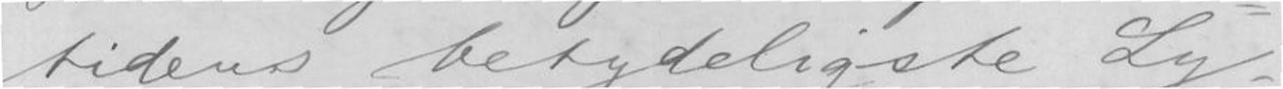tidens betydeligste Ly-
